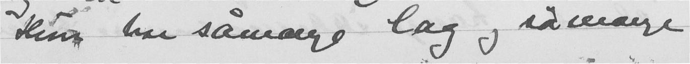Hun har såmange lag og såmange
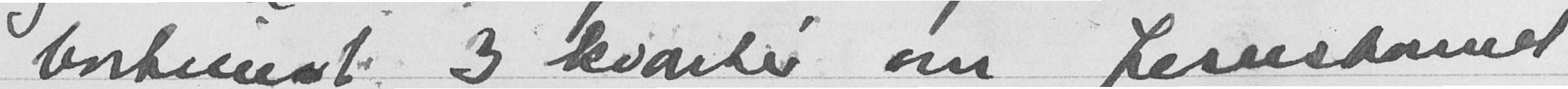bortimot 3 kvarter om Jesusbarnet
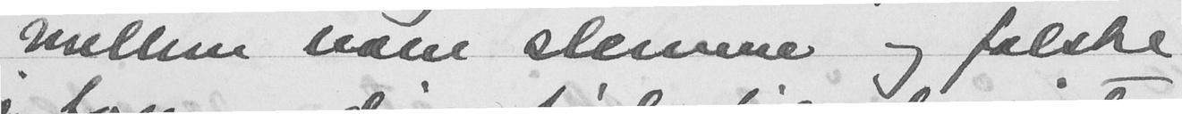mellom noen slemme og falske
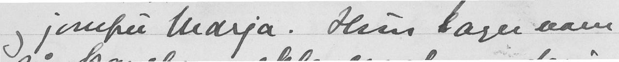og jomfru Maria. Hun lager som
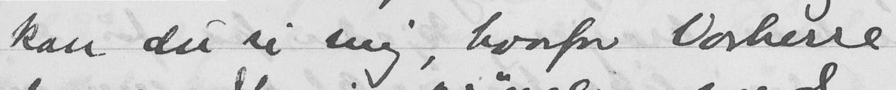kan du si mig, hvorfor Vorherre
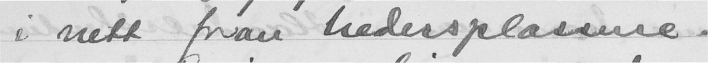i nett foran hedersplassene.
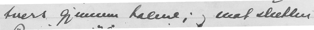tvers gjennem talene; og mat slitten
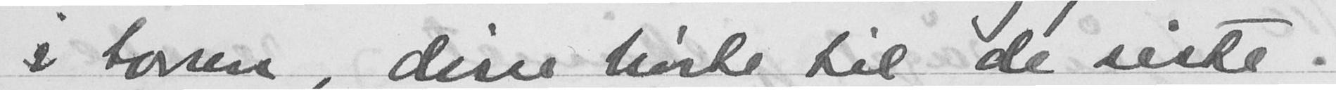i tonen, disse hørte til de siste.
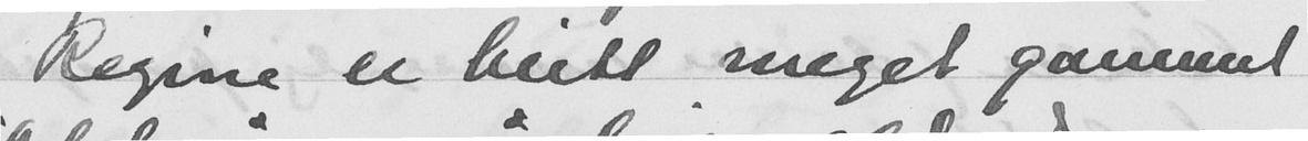Regine er blitt meget gammel
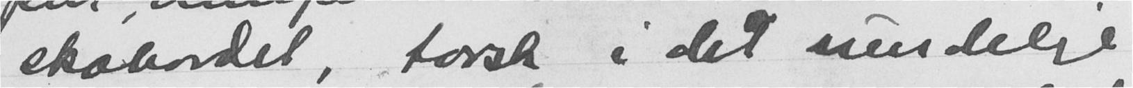skobordet, torsk i det uendelige
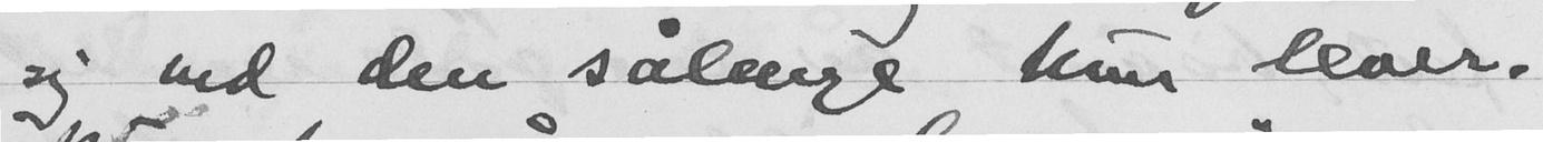sig ved den sålenge hun lever.
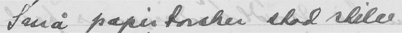Små papirtorsker stod stilt
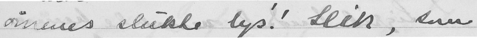øinenes slukte lys. Slik, som
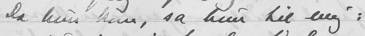Da hun kom, sa hun til mig:
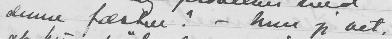denne fæsten? - Men jeg vet,
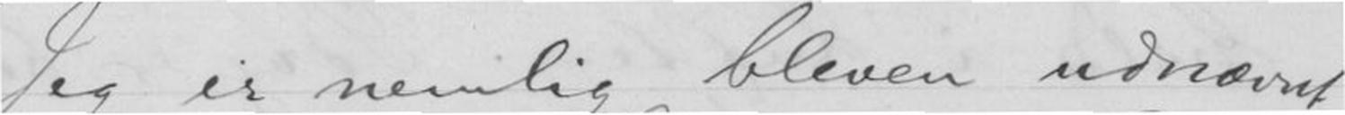Jeg er nemlig bleven udnævnt
